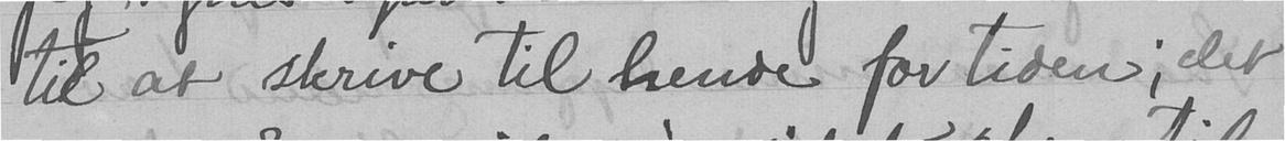til at skrive til hende fortiden; det
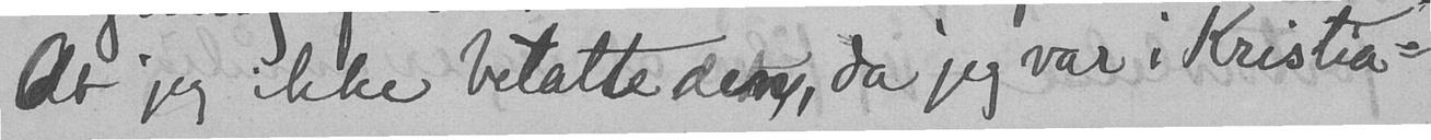At jeg ikke betalte dem, da jeg var i Kristia-
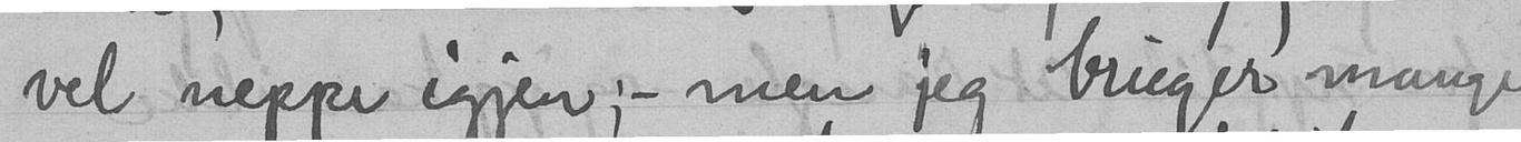vel neppe igjen; - men jeg bruger mange
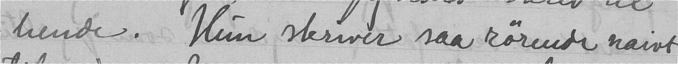hende. Hun skriver saa rørende naivt
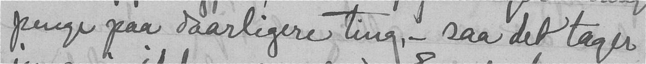penge paa daarligere ting, - saa det tager
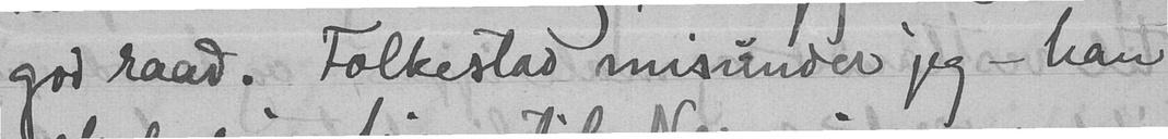god raad. Folkestad misunder jeg - han
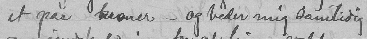et par kroner - og beder mig samtidig
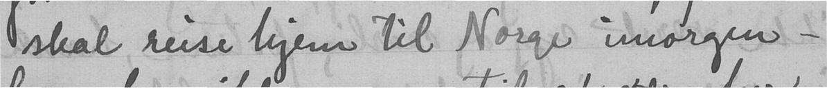skal reise hjem til Norge imorgen -
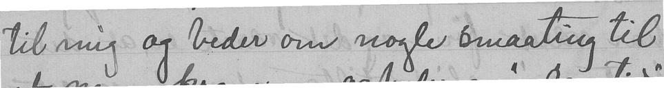til mig og beder om nogle smaating til
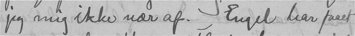jeg mig ikke nær af. - Engel har faaet
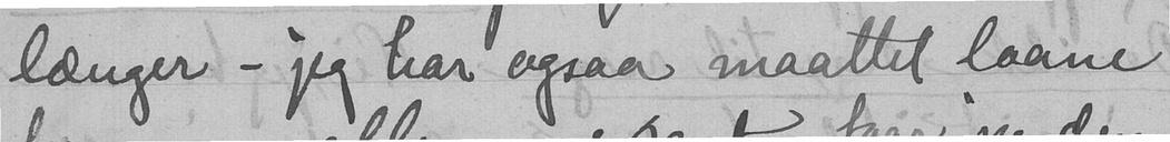længer: - jeg har ogsaa maattet laane
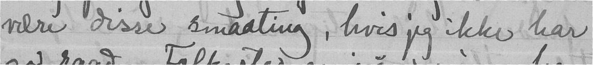være disse smaating, hvis jeg ikke har
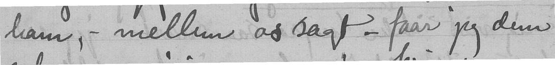ham, - mellem os sagt - faar jeg dem
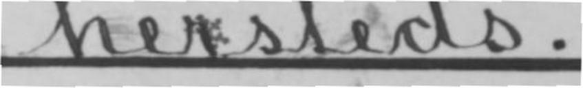hersteds.
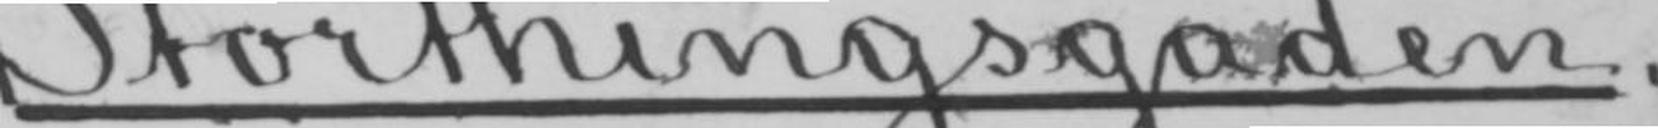Storthingsgaden,
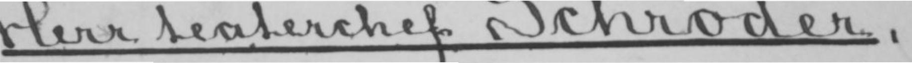Herr teaterchef Schrøder,
